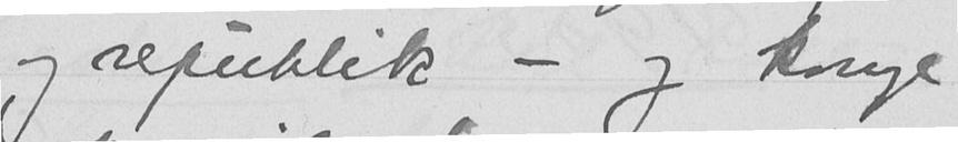og republik - og konge
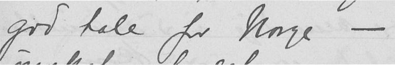god tale for Norge -
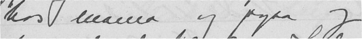hos mama og papa og
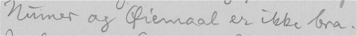Numer og Øiemaal er ikke bra.
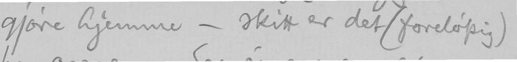gjøre hjemme - skitt er det (foreløpig)
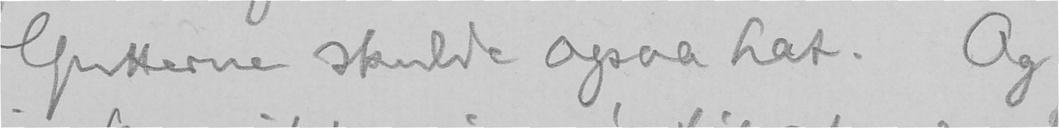Gutterne skulde ogsaa hat. Og
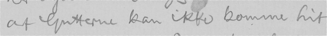at Gutterne kan ikke komme hit
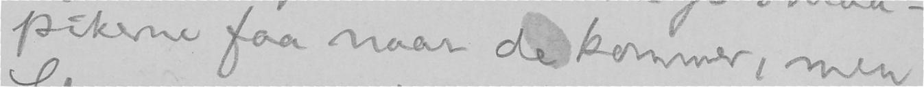pikerne faa naar de kommer, men
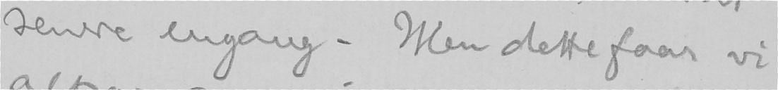senere engang. Men dette faar vi
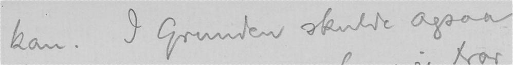kan. I Grunden skulde ogsaa
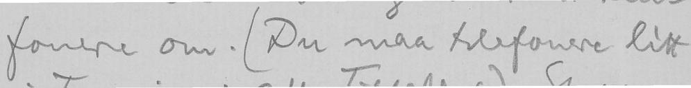fonere om. (Du maa telefonere litt
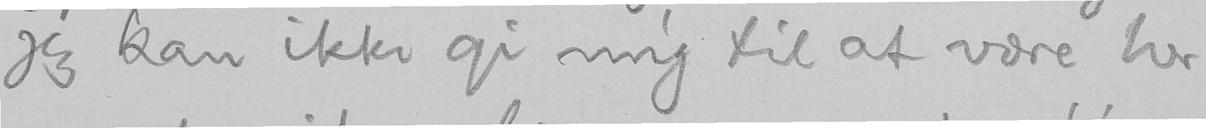jeg kan ikke gi mig til at være her
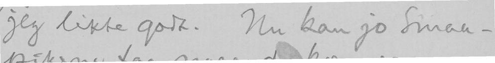jeg likte godt. Nu kan jo Smaa-
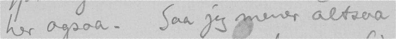her ogsaa. Saa jeg mener altsaa
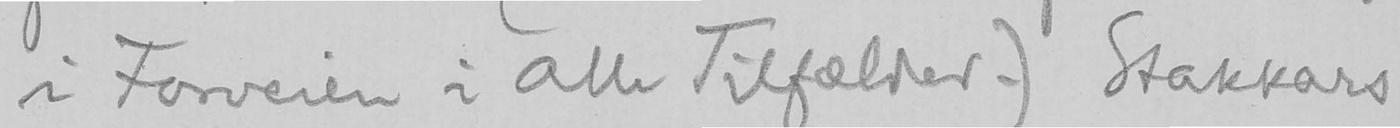i Forveien i alle Tilfælder.) Stakkars
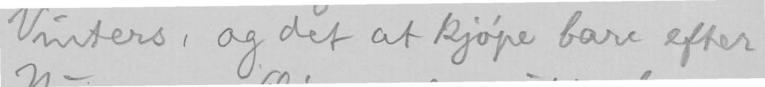Vinters, og det at kjøpe bare efter
